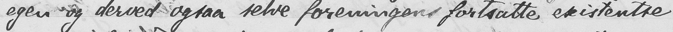egen og derved ogsaa selve foreningens fortsatte existentse
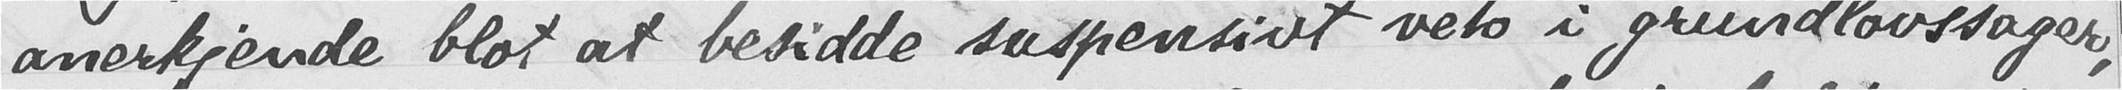anerkjende blot at besidde suspensivt veto i grundlovssager,
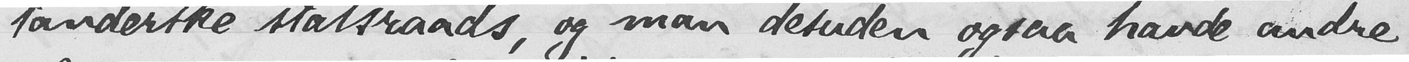tanderske statsraads, og man desuden ogsaa havde andre
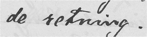de retning.
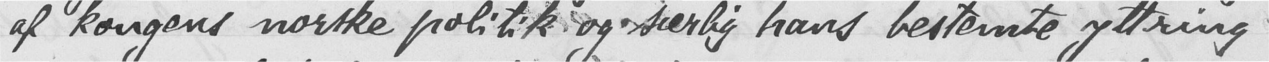af kongens norske politik og særlig hans bestemte yttring
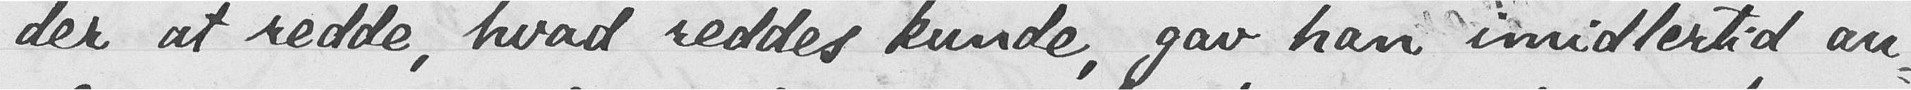der at redde, hvad reddes kunde, gav han imidlertid an-
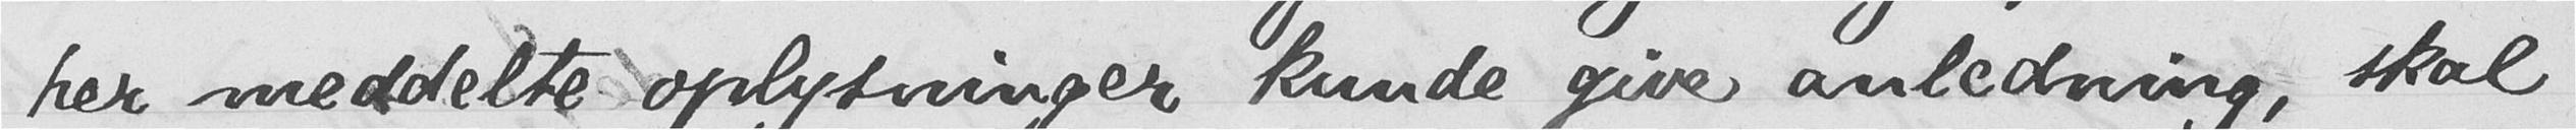her meddelte oplysninger kunde give anledning, skal
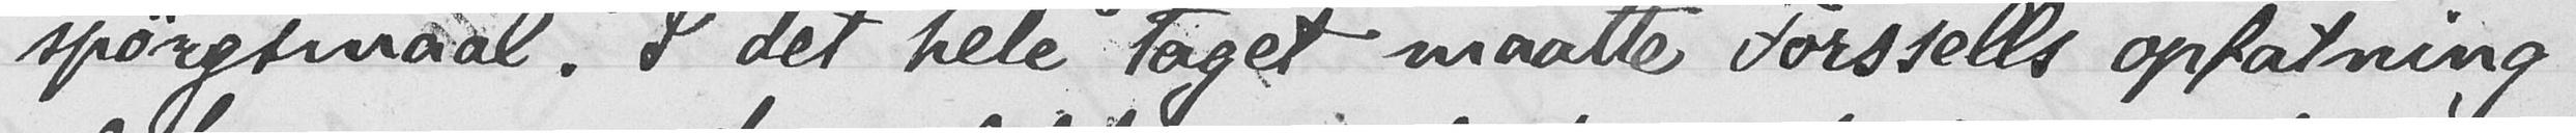spørgsmaal. I det hele taget maatte Forssells opfatning
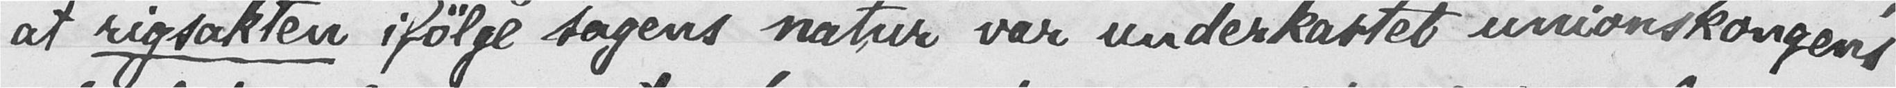at rigsakten ifølge sagens natur var underkastet unionskongens
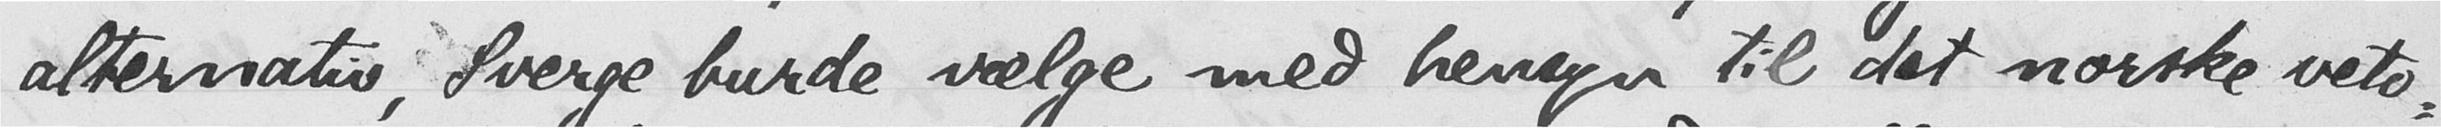alternativ, Sverge burde vælge med hensyn til det norske veto-
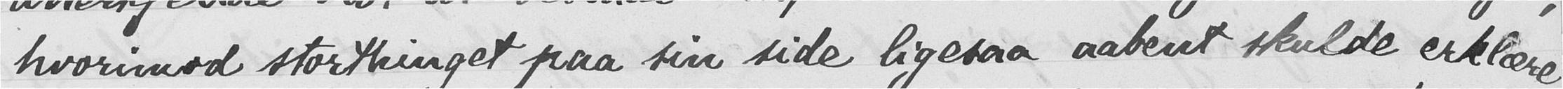hvorimod storthinget paa sin side ligesaa aabent skulde erklære,
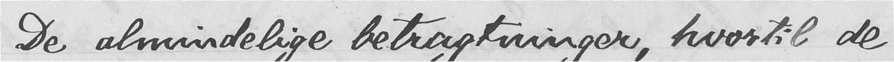De almindelige betragtninger, hvortil de
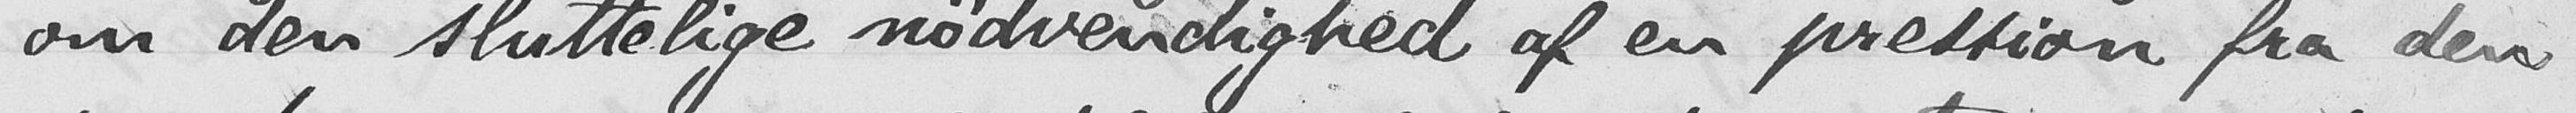om den sluttelige nødvendighed af en pression fra den
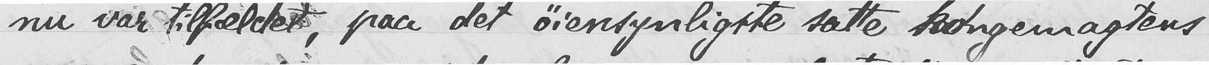nu var tilfældet, paa det øiensynligste satte kongemagtens
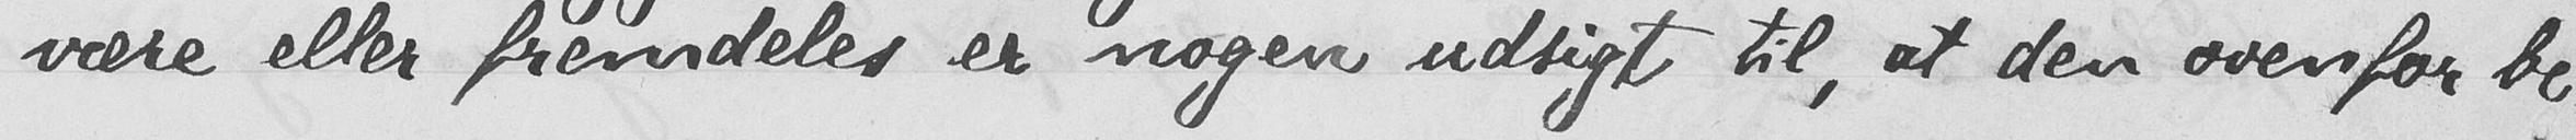være eller fremdeles er nogen udsigt til, at den ovenfor be-
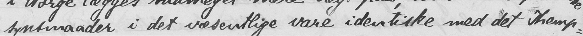synsmaader i det væsentlige være identiske med det Themp-
